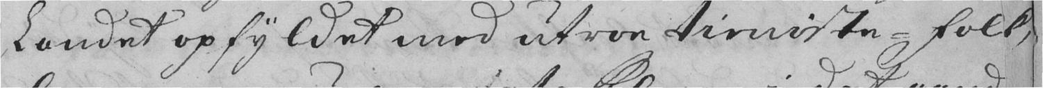Landet opfyldet med utroe tieniste-Folk,
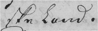ske Land.
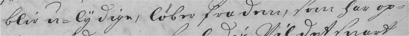blev u-lydige, løber fra dem, som har op-
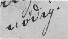nødig.
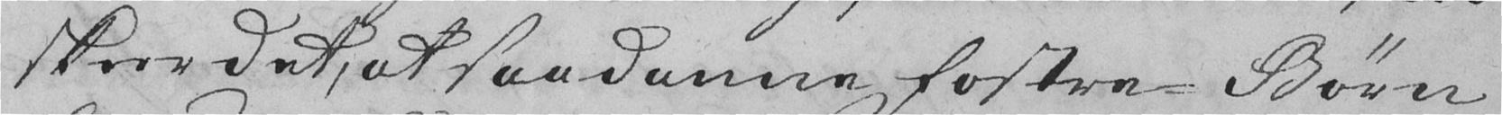skeer det, at saadanne Foster-Børn
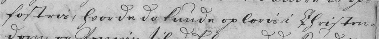fostris, hvor de da kunde oplæris i Christen-
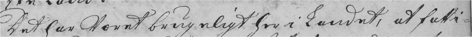Det har været brugeligt her i Landet, at fatti-
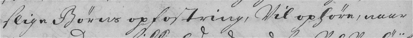slige Børns opfostring, Vil ophøre, naar
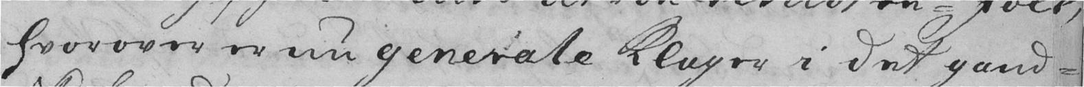hvorover er nu generale Klager i det gand-
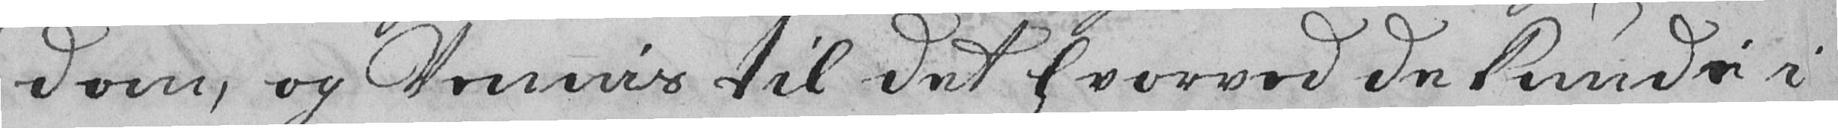dom, og Vennis til det hvorved de kunde i
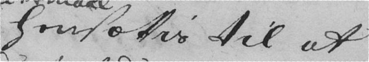hensættis til at
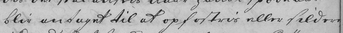blir antaget til at opfostris eller sildere
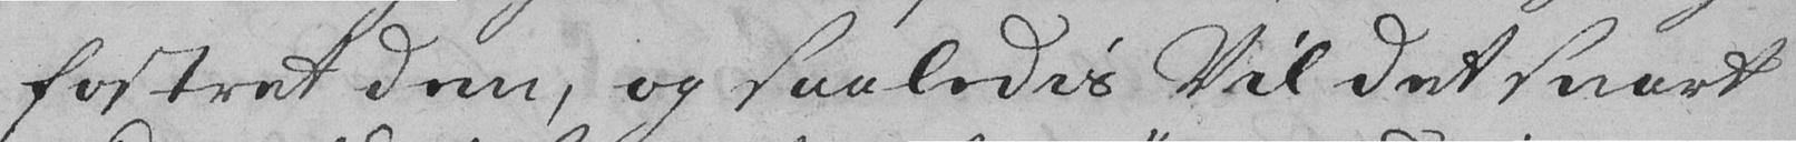fostret dem, og saaledis Vil det snart
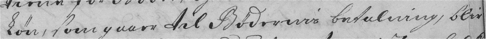Løn, som gaaer til Bødernis betaling, blir
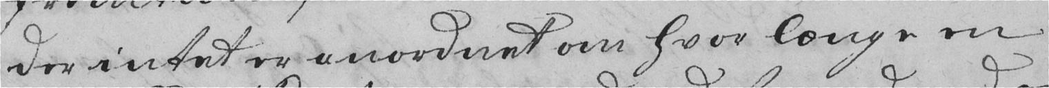der intet er anordnet om hvor længe en
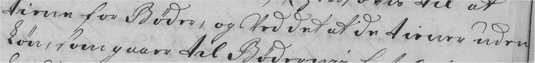tiene for Bøder, og Ved det at de tiener uden
